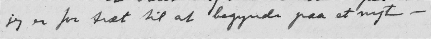jeg er for træt til at begynde paa et nyt -
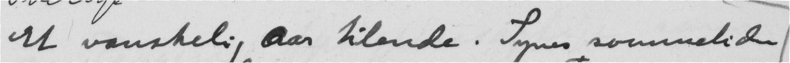Et vanskelig Aar tilende. Synes sammetiden
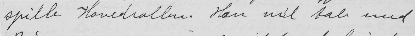spille Hovedrollen. Han vil tale med
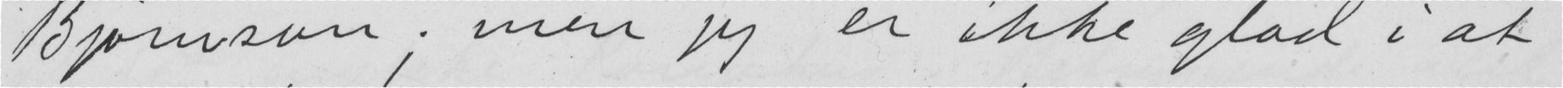Bjørnson; men jeg er ikke glad i at
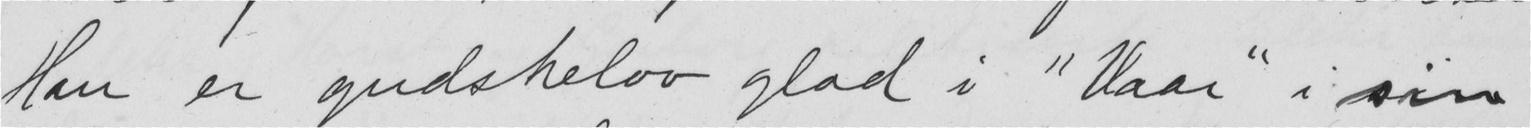Han er gudskelov glad i "Vaar" i sin
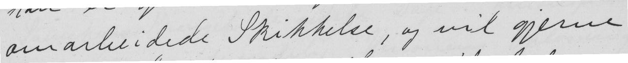omarbeidede Skikkelse, og vil gjerne
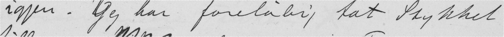igjen. Jeg har foreløbig tat Stykket
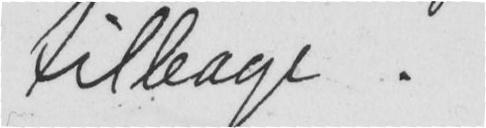tilbage.
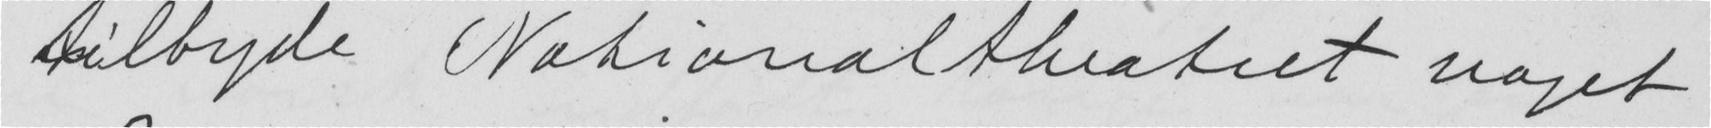tilbyde Nationaltheatret noget
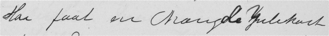Har faat en Mængde Julekort
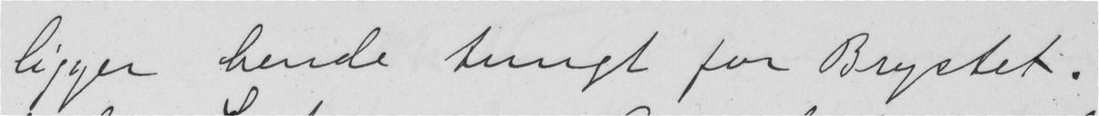ligger hende tungt for Brystet.
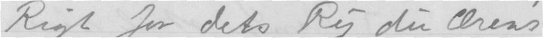Rigt for dets Ry du Ærens
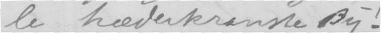le hæderkranste By!
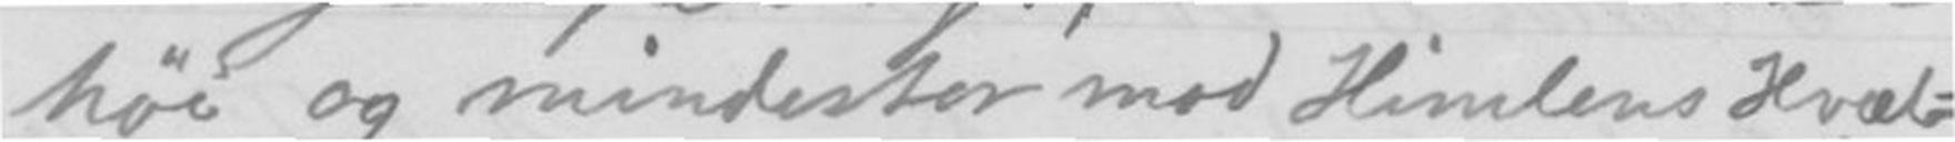høi og mindestor mod Himlens Hvæl
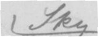Sky
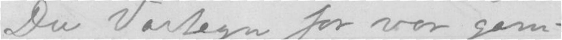Du Vartegn for vor gam-
